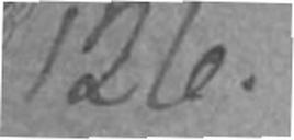126.
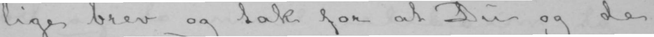lige brev og tak for at Du og de
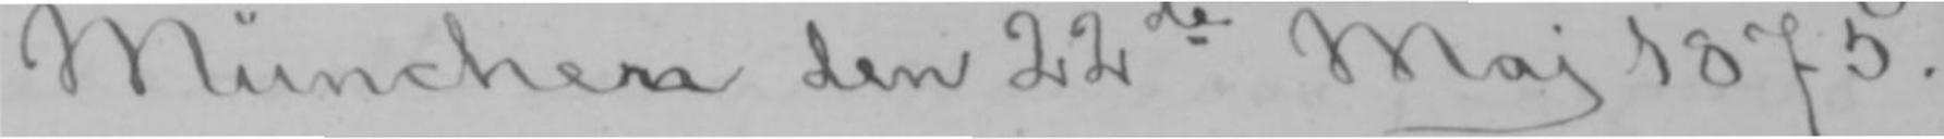München den 22de Maj 1875.
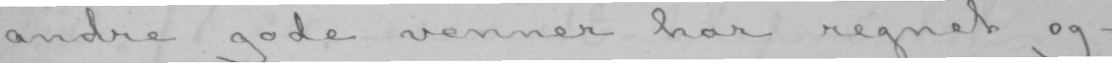andre gode venner har regnet og-
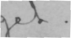get.
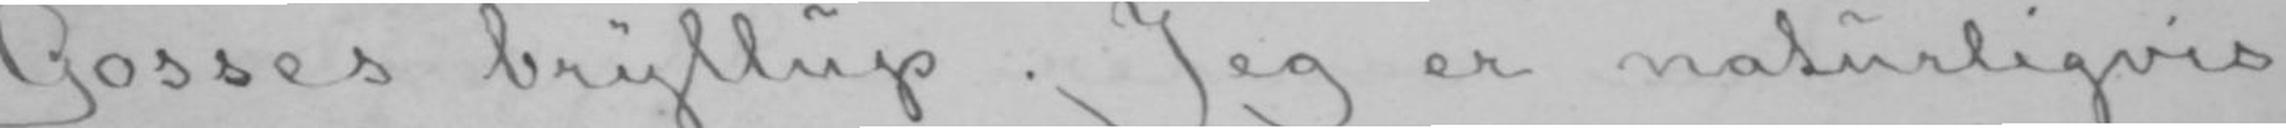Gosses bryllup. Jeg er naturligvis
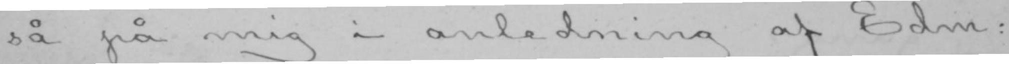så på mig i anledning af Edm:
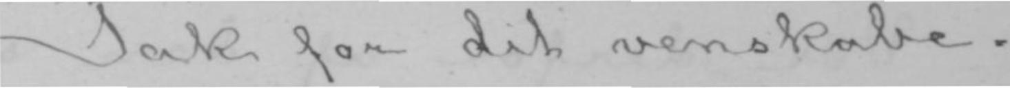Tak for dit venskabe-
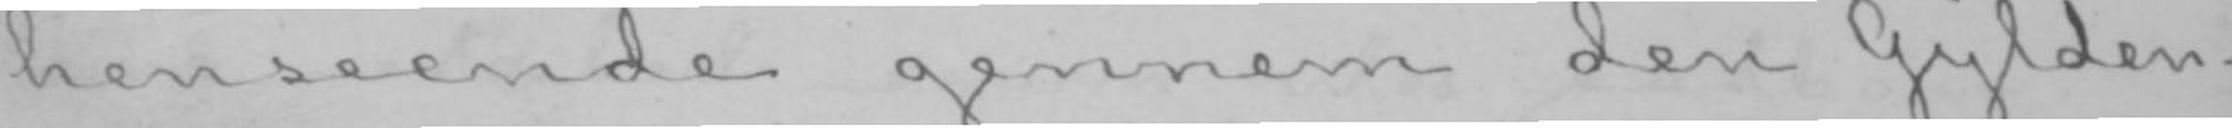henseende gennem den Gylden-
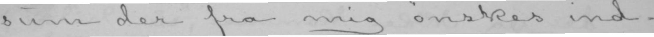sum der fra mig ønskes ind-
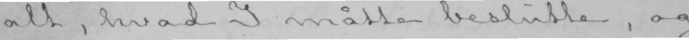alt, hvad I måtte beslutte, og
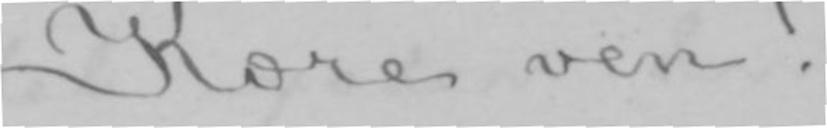Kære ven!
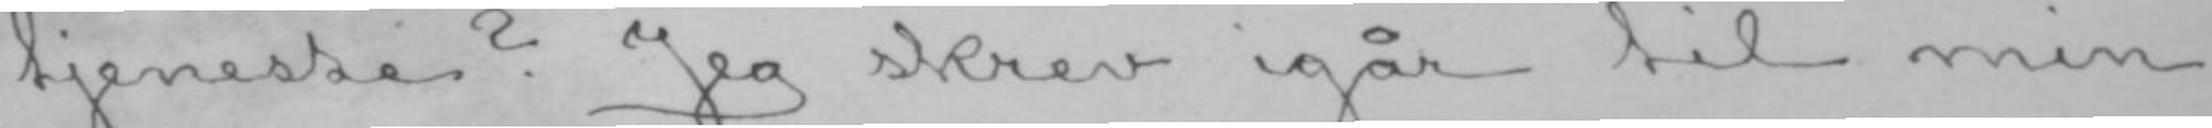tjeneste? Jeg skrev igår til min
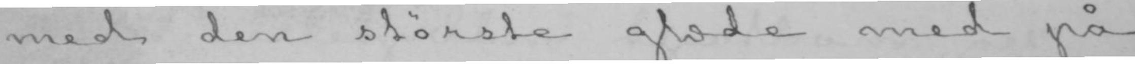med den største glæde med på
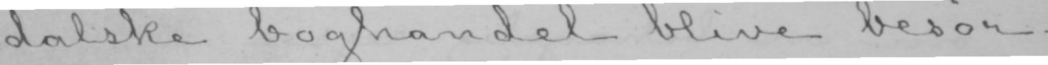dalske boghandel blive besør-
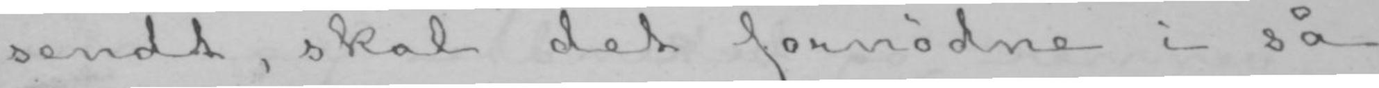sendt, skal det fornødne i så
